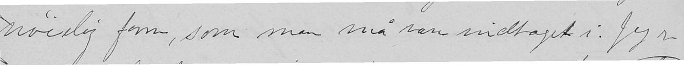nøielig form, som man må være indtaget i. Jeg er
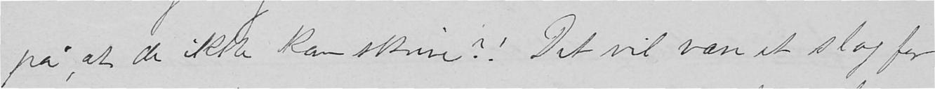på at de ikke kan skrive?! Det vil være et slag for
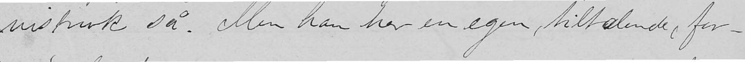vistnok så. Men han har en egen, tiltalende, for-
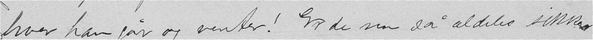hvor han går og venter! Er de nu så aldeles sikker
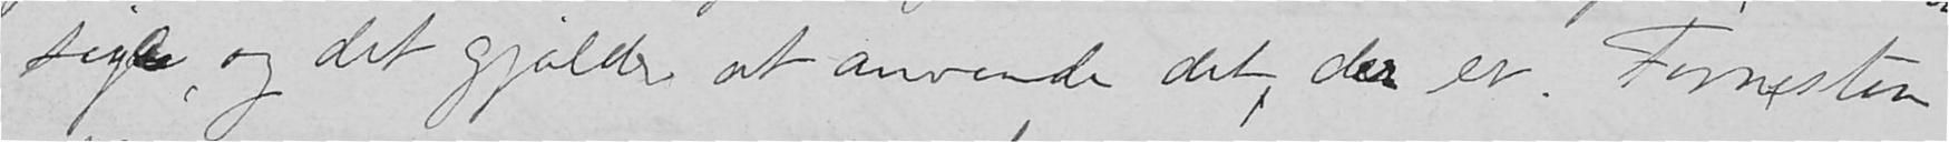sige, og det gjelder at anvende det, der er. Forresten
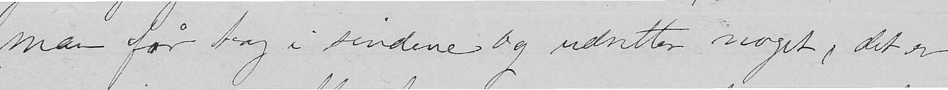man får tag i sindene og udretter noget, det er
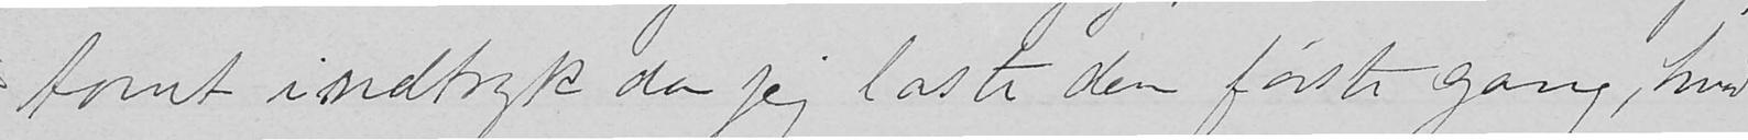tomt indtryk da jeg læste den første gang, hvad
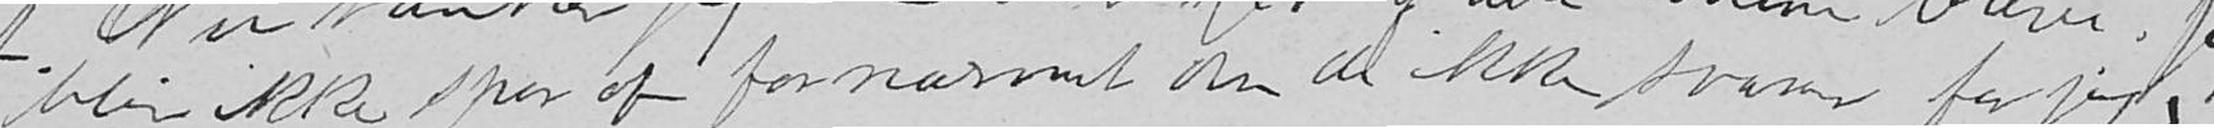blir ikke spor af fornærmet om de ikke svare for jeg
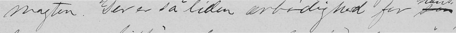magten. Der er så liden arbødighed for sin
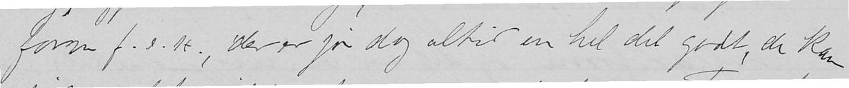form f.e.x., der er jo dog altid en hel del godt, de kan
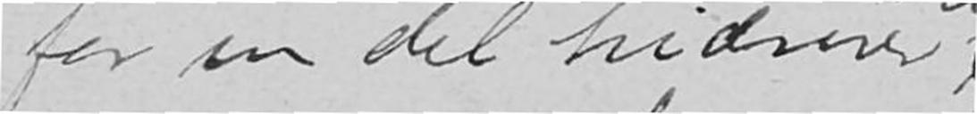for en del hidrører
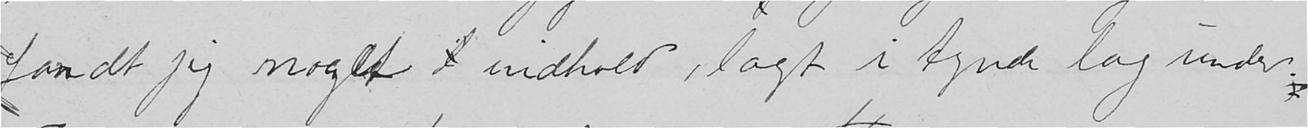fandt jeg noget i indhold, lagt i tynde lag under.
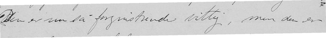Den er nu så forgnistrende vittig, men den er
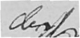der-
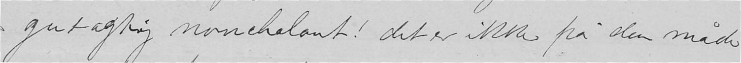gutagtig nonchalant! det er ikke på den måde
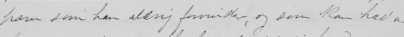ham som han aldrig forvinder, og som kan hae' u-
